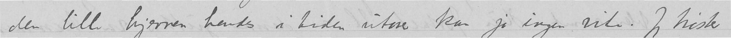den lille hjernen hendes i tiden utover kan jo ingen vite. Jeg trøster
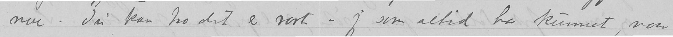noe. Du kan tro det er rart – jeg som altid ha kunnet, naar
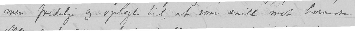men fredelige og oplagte til at være snille mot hverandre.
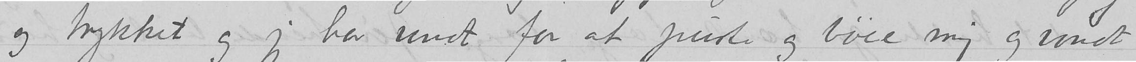og trykket og jeg har vondt for at puste og bøie mig og vondt
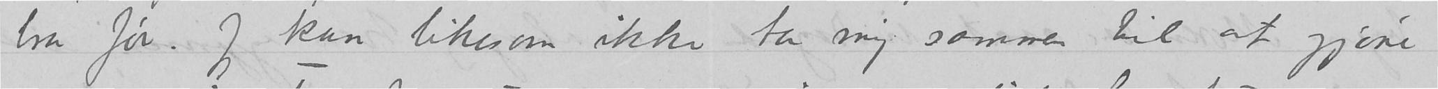bra før. Jeg kan likesom ikke ta mig sammen til at gjøre
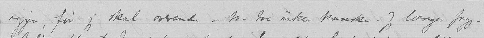igjen, før jeg skal overende – to – tre uker kanske. Jeg længes fryg-
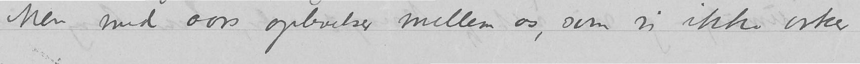Men med aars oplevelser mellem os, som vi ikke orker
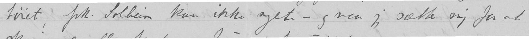tøiet, frk. Solheim kan ikke sylte – og naar jeg sætter mig for at
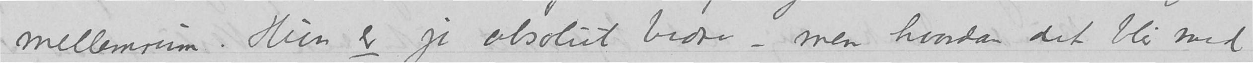mellemrum. Hun er jo absolut bedre – men hvordan det blir med
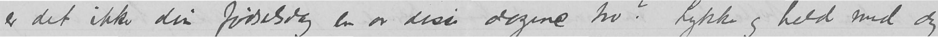er det ikke din fødselsdag en av disse dagene tro? Lykke og held med dig,
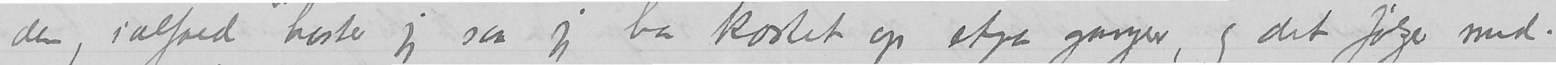den, ialfald hoster jeg saa jeg har kastet op etpar ganger, og det følger med.
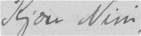Kjære Nini
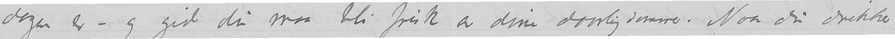dagen er – og gid du maa bli frisk av dine daarligsomre Naar du drikker
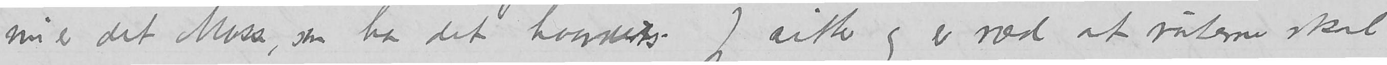nu er det Mosse, som har det haardests.  Jeg sitter og er ræd at vinduerne ruterne skal
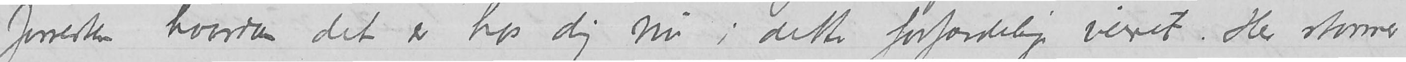forresten hvordan det er hos dig nu i dette forfærdelige veiret. Her stormer
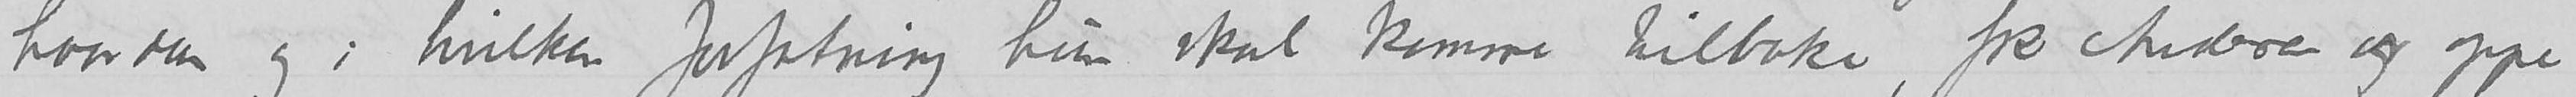hvordan og i hvilken forfatning hun skal komme tilbake, frk. Andersen er oppe
